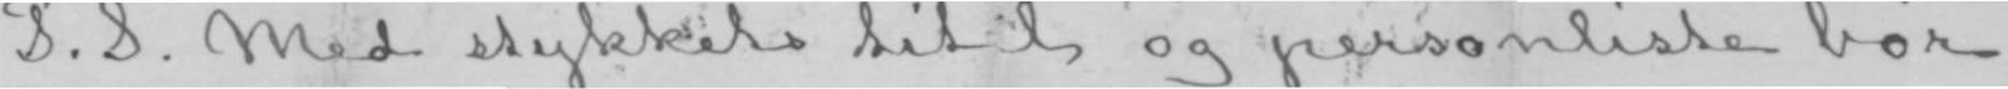P. S. Med stykkets titel og personliste bør
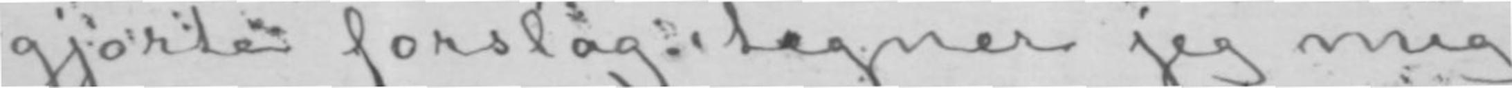gjorte forslag tegner jeg mig
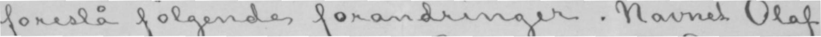foreslå følgende forandringer. Navnet Olaf
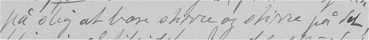på slig at bare stirre og stirre på det,
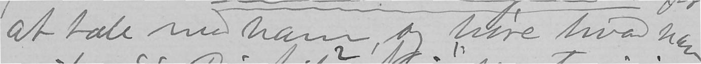at tale med ham, og høre hvad han
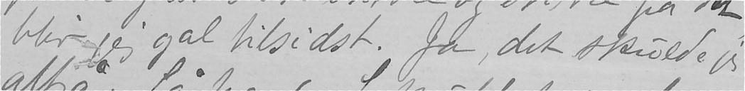blir jeg gal tilsidst. Ja, det skulde jeg
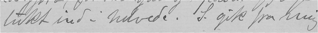lukt ind i helvede. S. gik fra mig
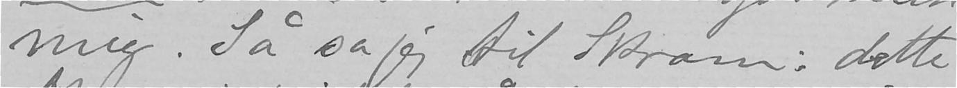mig. Så sa jeg til Skram: dette
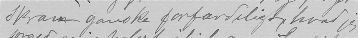Skram ganske forfærdeligt, hvad jeg
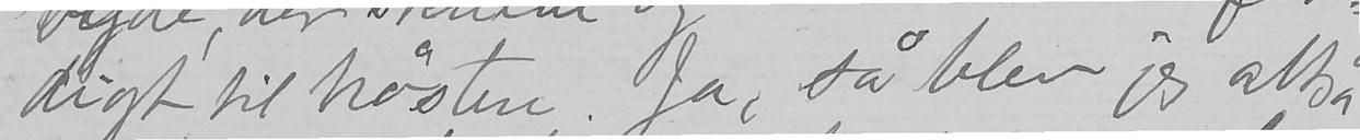digt til høsten. Ja, så blev jeg altså
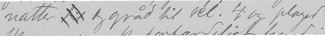nætter til og gråd til kl. 4 og plaged
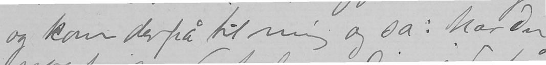og kom derpå til mig og sa: har Du
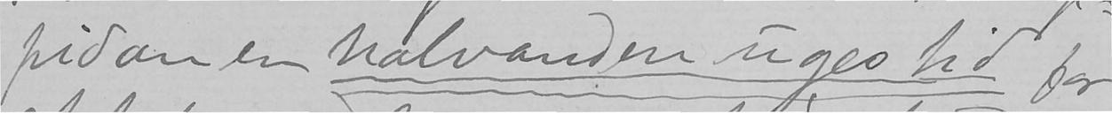pidan en halvanden uges tid for
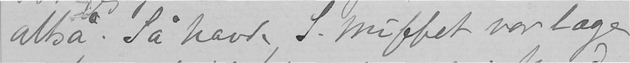altså. Så havde S. truffet vor læge
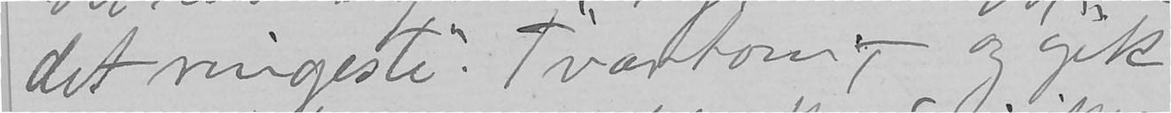det ringeste". Tværtom,- og gik
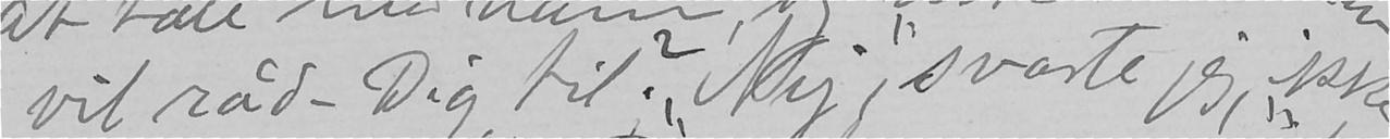vil råde Dig til? "Nej," svarte jeg, "ikke
